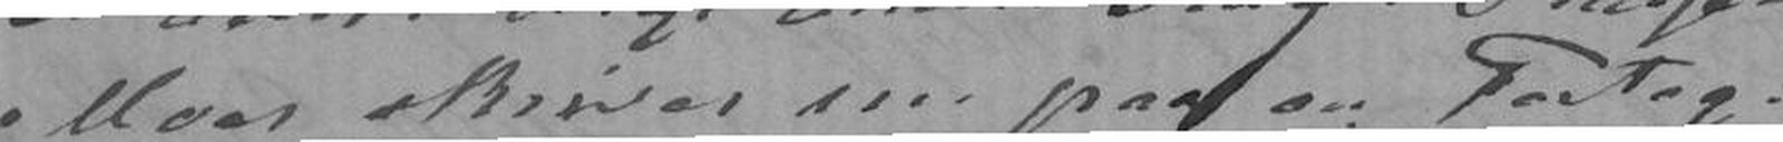Moer skriver nu paa en Forteg-
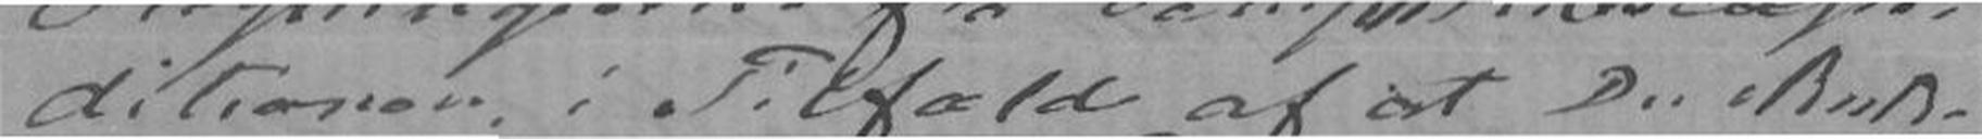ditionen, i Tilfælde af at Du skulde
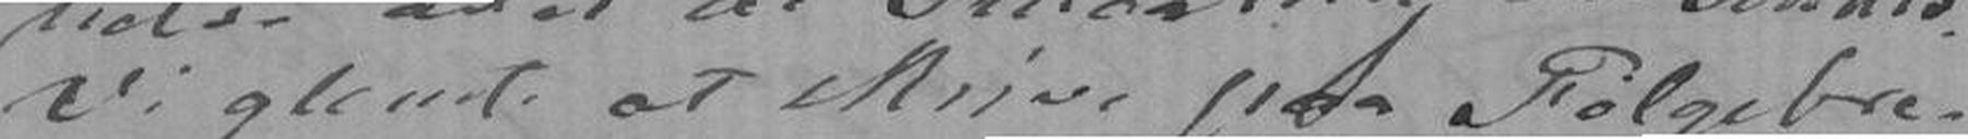Vi glemte at skrive paa Følgebre-
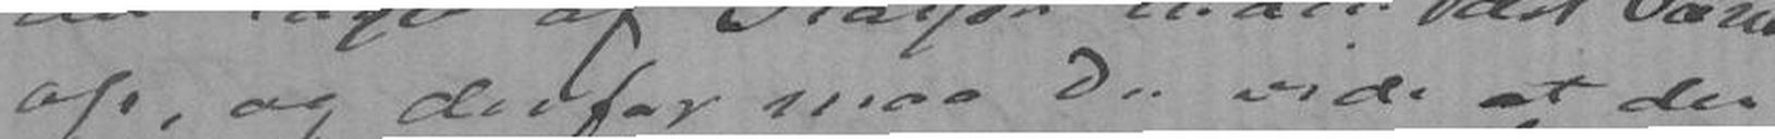op. og derfor maa Du vide at der
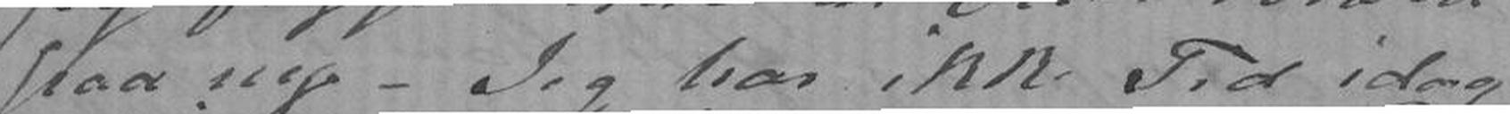paa ny. - Jeg har ikke Tid idag
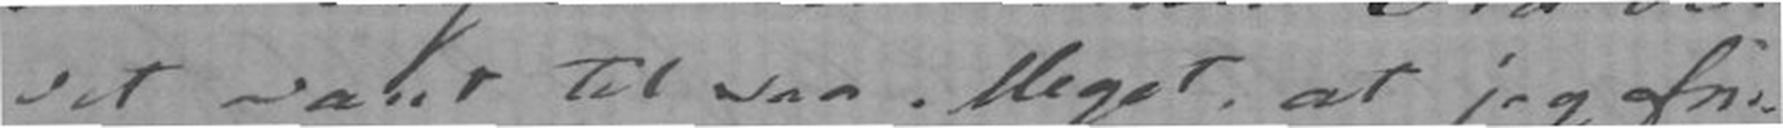vet vant til saa Meget, at jeg fin-
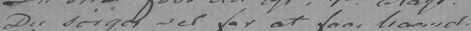Du sørger vel for at faae haand-
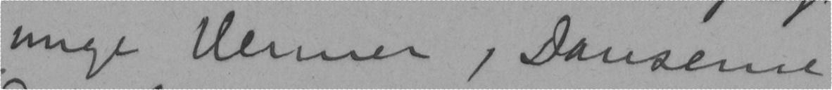unge Venner, Danserne
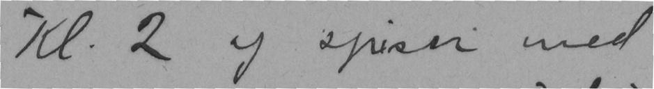Kl. 2 og spiser med
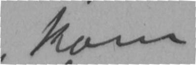kom
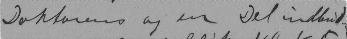Doktorens og en Del indbud-
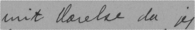mit Værelse da jeg
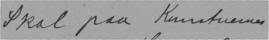Skal paa Kunstnernes
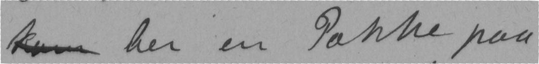her en Pakke paa
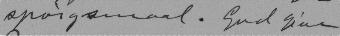spørgsmaal. Gud give
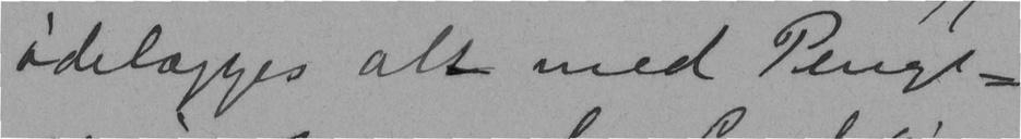ødelægges alt med Penge-
